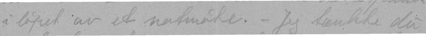i løpet av et natmøte. - Jeg tænkte du
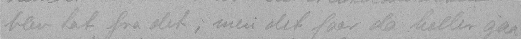blev tat fra det; men det faar da heller gaa
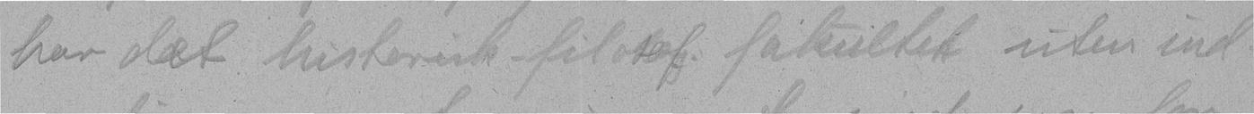har det historisk-filosof. fakultet uten ind-
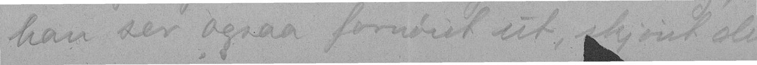han ser ogsaa fornøiet ut, skjønt det jo
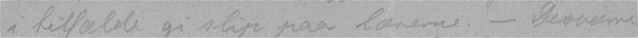i tilfælde gi slip paa lærerne. - Desverre
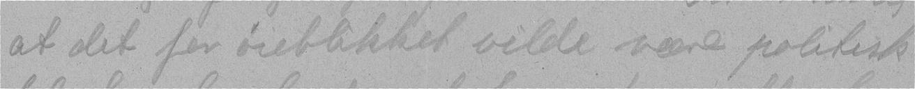at det for øieblikket vilde være politisk
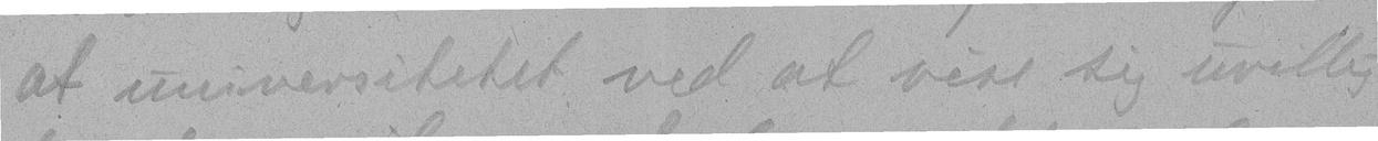at universitetet ved at vise sig uvillig
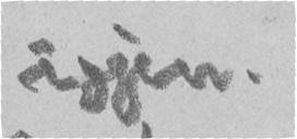igjen.
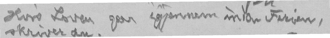Hvis Loven gar igjennem inden Ferien,
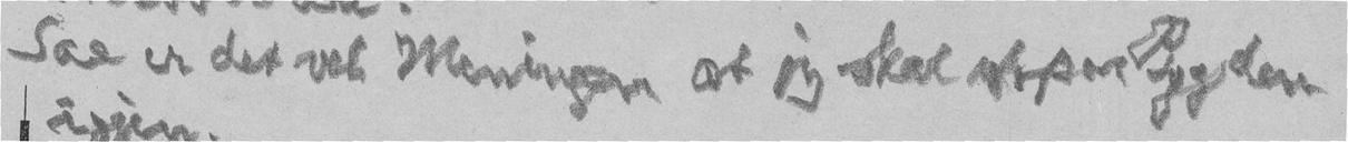Saa er det vel Meningen at jeg skal utpaa Bygden
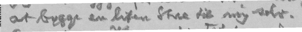at bygge en liten Stue til mig selv.
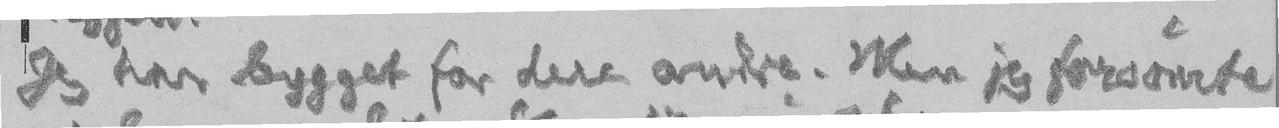Jeg har bygget for dere andre. Men jeg forsømte
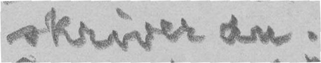skriver du.
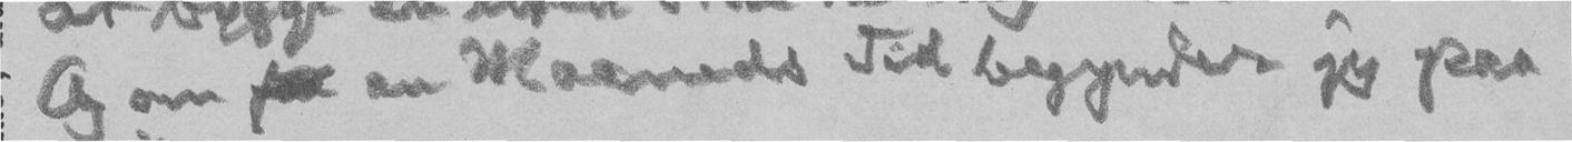Og om fik en Maaneds Tid begynder jeg paa
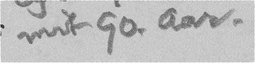mit 90. Aar.
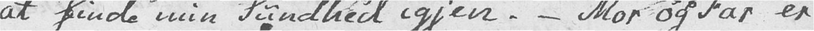at finde min Sundhed igjen. – Mor og Far er
